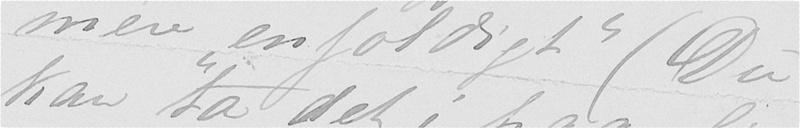mere enfoldigt (Du
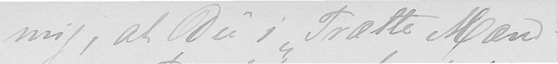mig, at Du i Trætte Mænd.
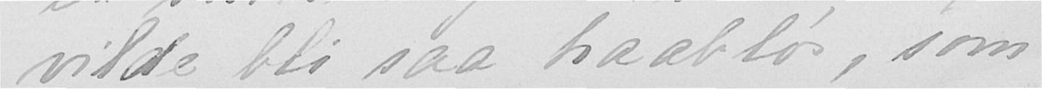vilde bli saa haabløs, som
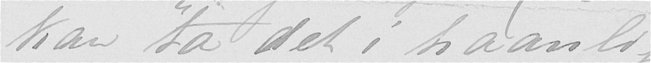kan ta det i haanlig
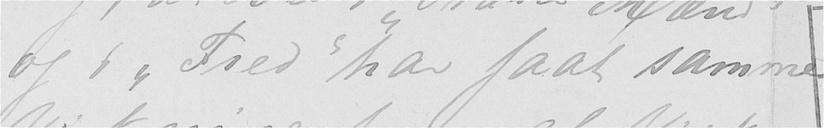og i Fred har faat samme
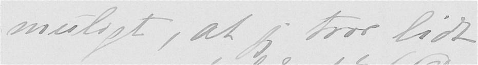muligt, at jeg tror lidt
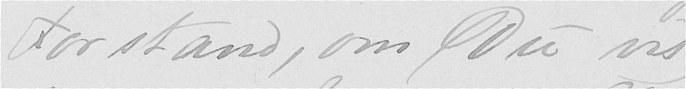Forstand, om Du vil)
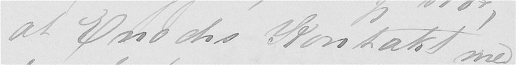at Enochs Kontakt med
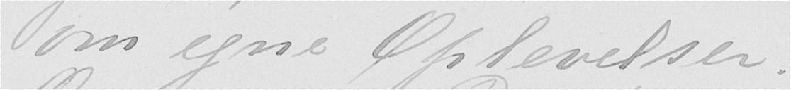som egne Oplevelser.
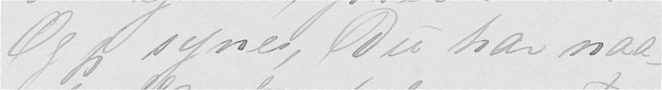Og jeg synes, Du har naa-
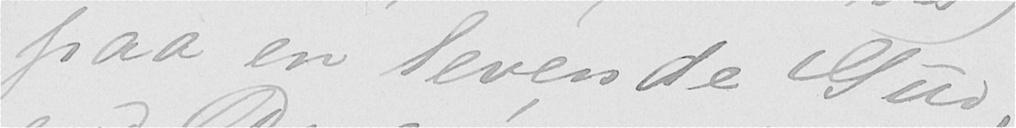paa en levende Gud,
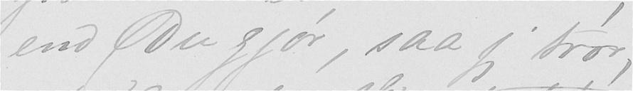end Du gjør, saa jeg tror,
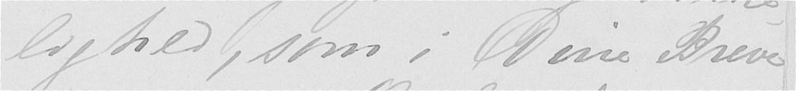lighed, som i Dine Breve
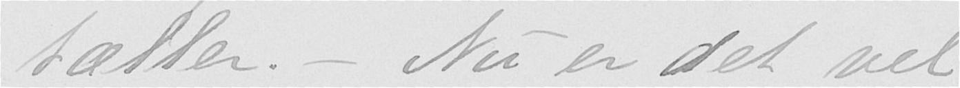tæller. - Nu er det vel
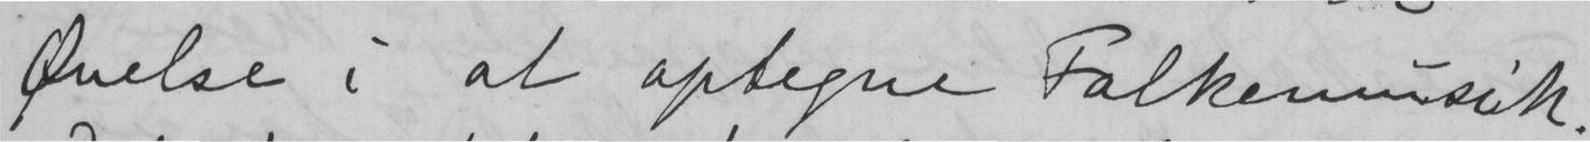Øvelse i at optegne Folkemusik.
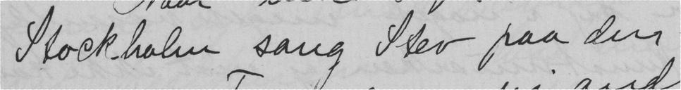Stockholm sang Stev paa den
